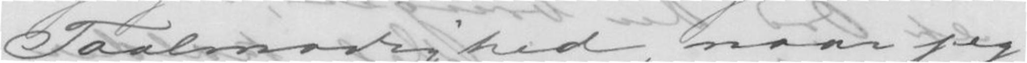Taalmodighed, naar jeg
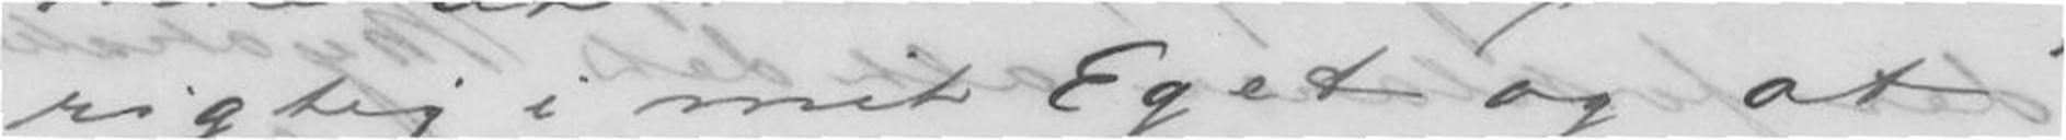rigtig i mit Eget og at
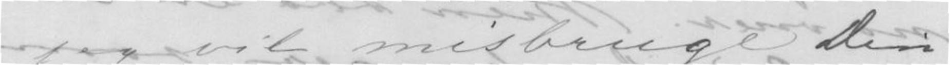jeg vil misbruge Din
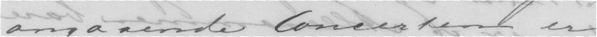angaaende Concerten er
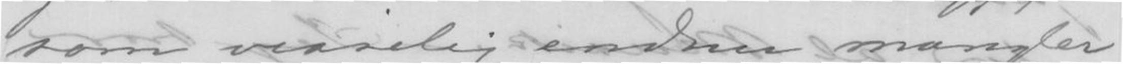som visselig endnu mangler
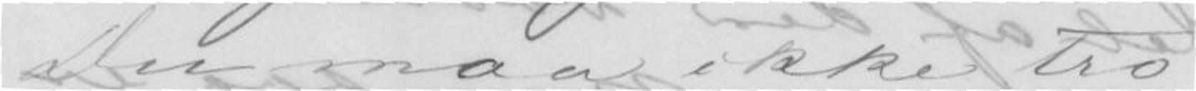Du maa ikke tro
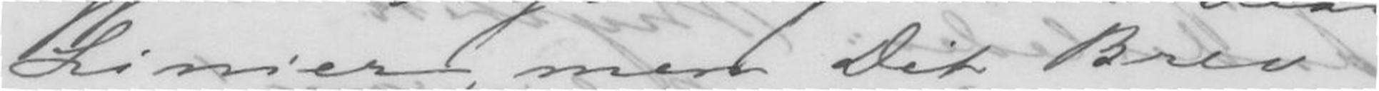Linier, men Dit Brev
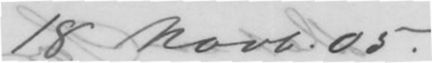18 Novb. 05.
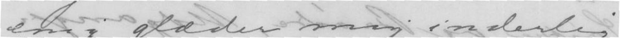enig glæder mig inderlig
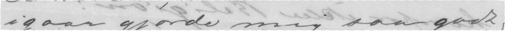igaar gjorde mig saa godt,
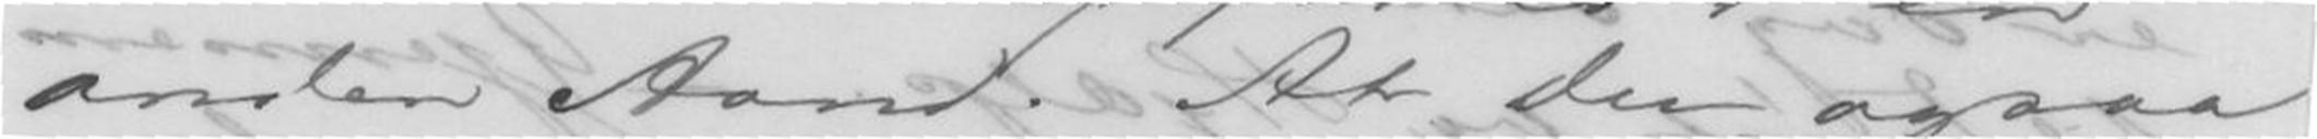anden Aand. At Du ogsaa
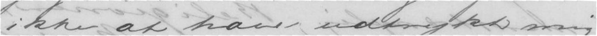ikke at have udtrykt mig
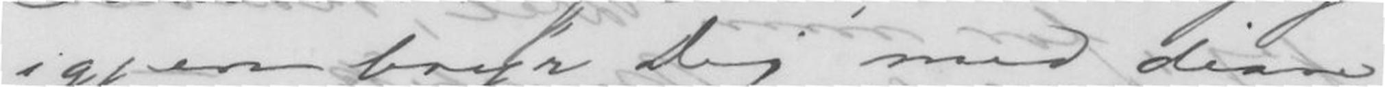igjen bryr Dig med disse
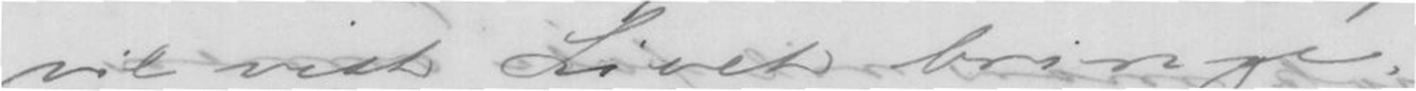vil vist Livet bringe.
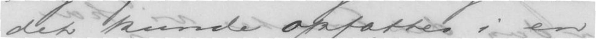det kunde opfattes i en
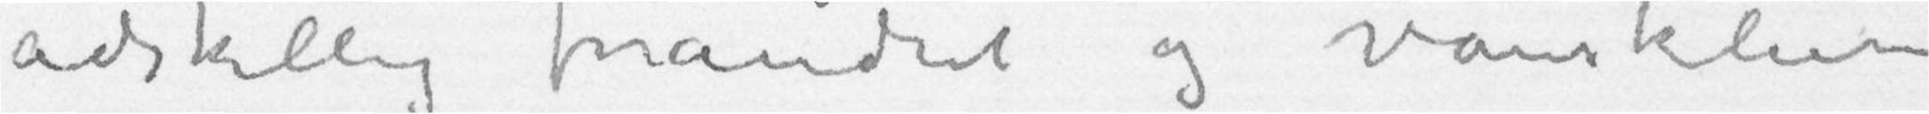adskillig forandret og vanskeliere
